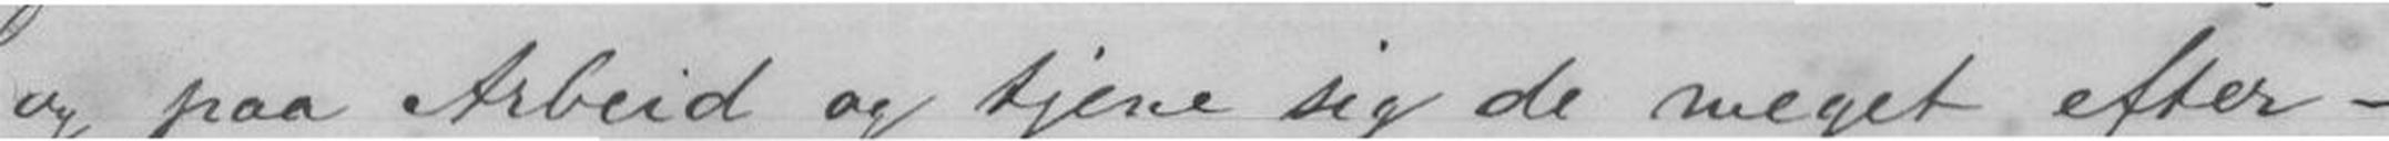og paa Arbeid og tjene sig de meget efter-
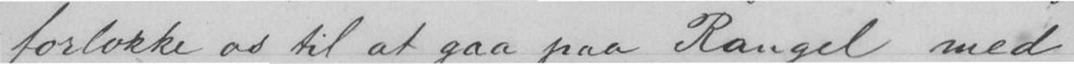forlokke os til at gaa paa Rangel med
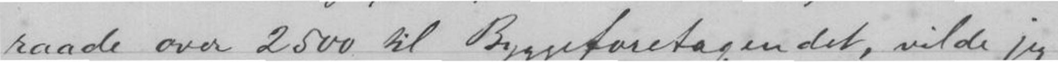raade over 2500 til Byggeforetagendet, vilde jeg
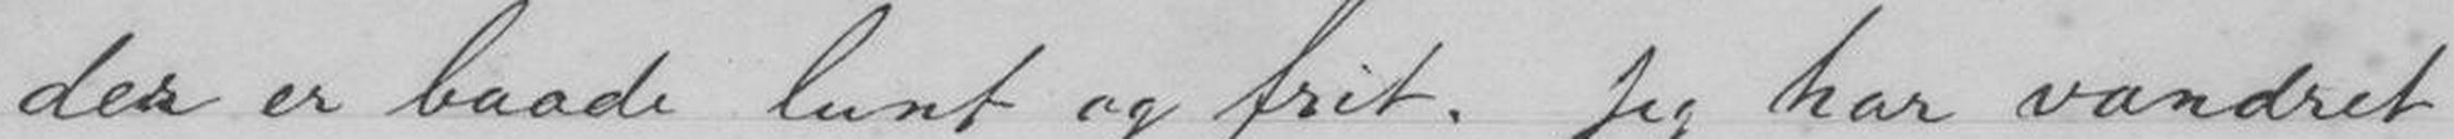der er baade lunt og frit. Jeg har vandret
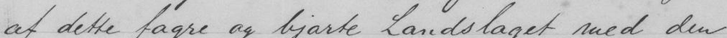af dette fagre og bjarte Landslaget med den
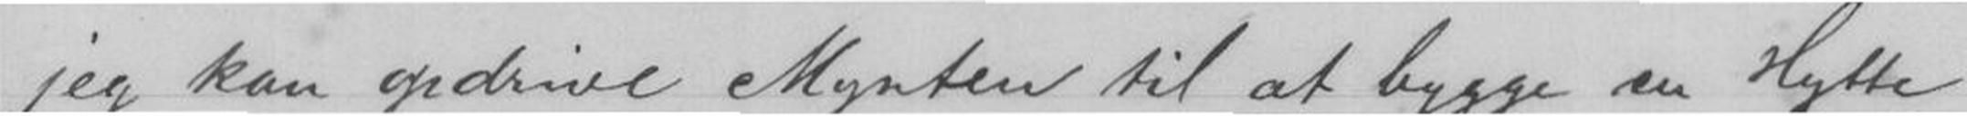jeg kan opgive Mynten til at bygge en Hytte
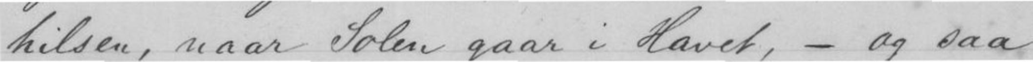hilsen, naar Solen gaar i Havet, - og saa
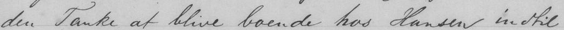den Tanke at blive boende hos Hansen indtil
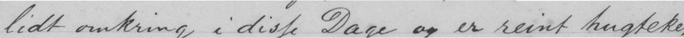lidt omkring i disse Dage og er reint hugteken
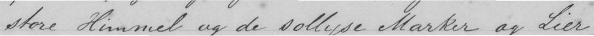store Himmel og de sollyse Marker og Lier
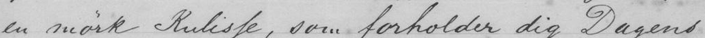en mørk Kulisse, som forholder dig Dagens
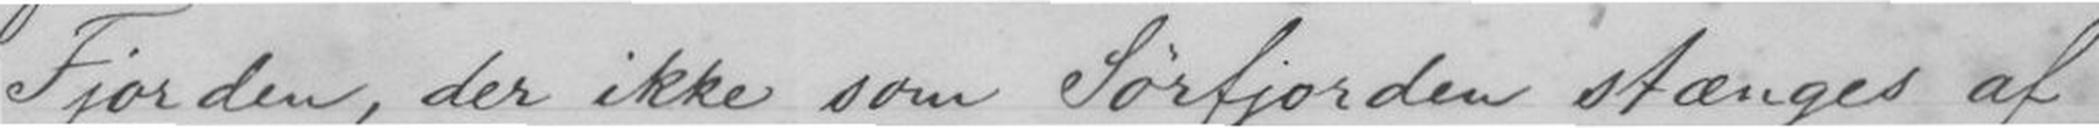Fjorden, der ikke som Sørfjorden stænges af
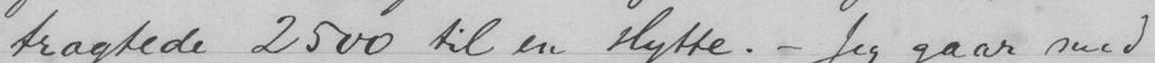tragtede 2500 til en Hytte. - Jeg gaar med
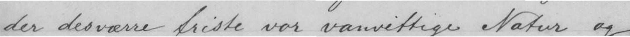der desværre friste vor vanvittige Natur og
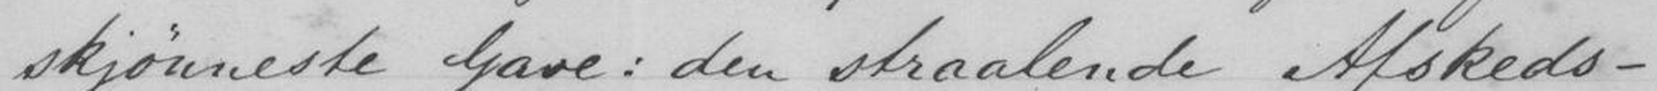skjønneste Gave: den straalende Afskeds-
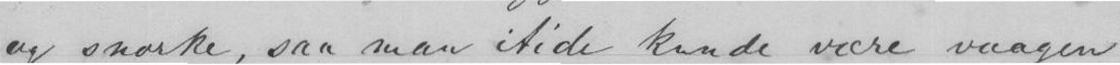og snorke, saa man itide kunde være vaagen
